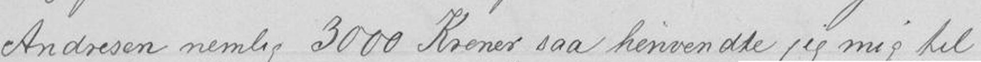Andresen nemlig 3000 Kroner, saa henvendte jeg mig til
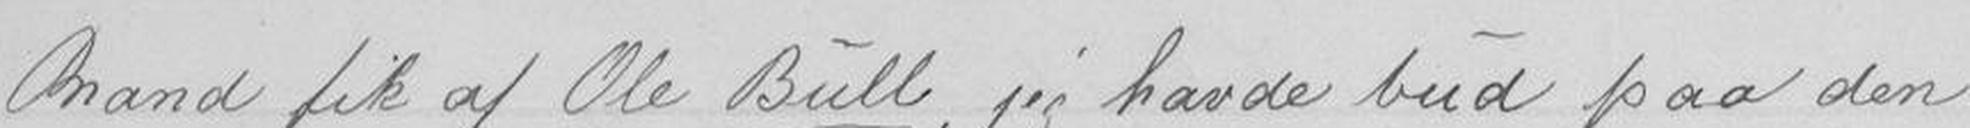Mand fik af Ole Bull, jeg havde bud paa den
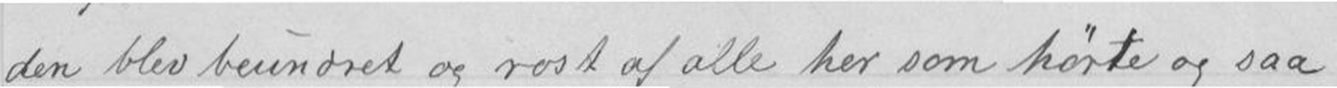den blev beundret og rost af alle her som hørte og saa
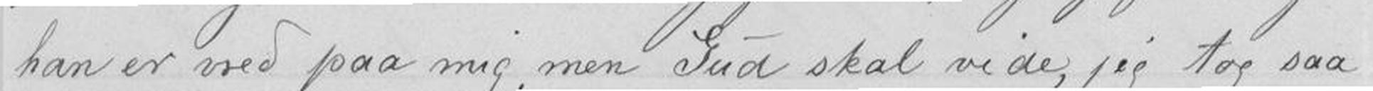han er vred paa mig, men Gud skal vide, jeg tog saa
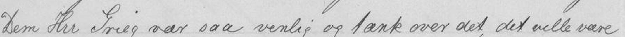Dem Hrr Grieg vær saa venlig og tænk over det, det ville være
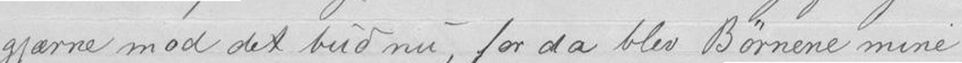gjærne mod det bud nu, for da blev Børnene mine
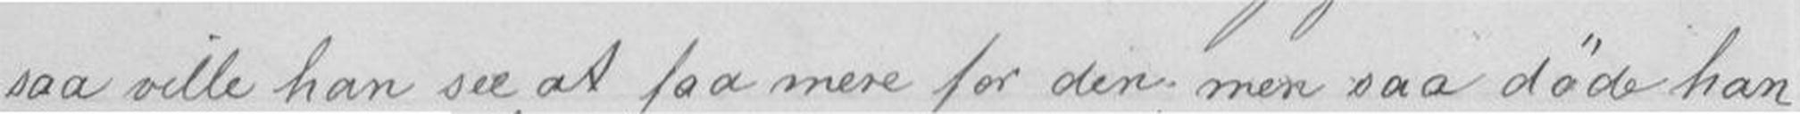saa ville han see, at faa mere for den, men saa døde han
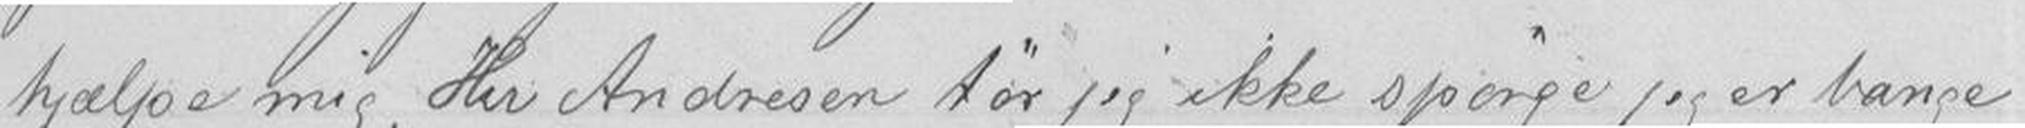hjælpe mig, Hrr Andresen tør jeg ikke spørge jeg er bange
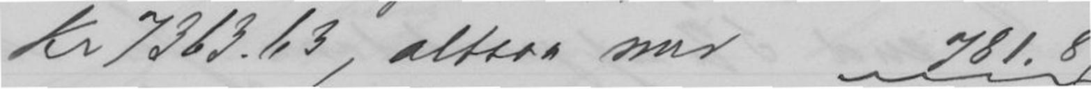kr 7363.63, altsaa med 781.87
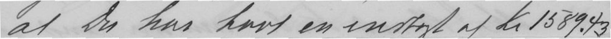at Du har havt en indtægt afkr 1589.43
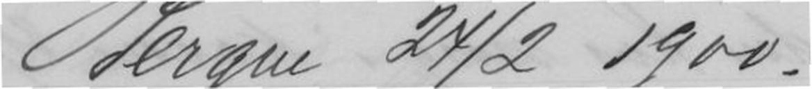Bergen 24/2 1900.
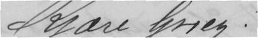Kjære Grieg!
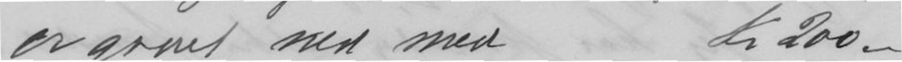er gaaet ned medkr 200.-
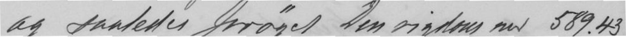og saaledes forøget Din rigdom med 589.43
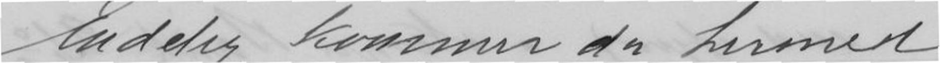Endelig kommer da hermed
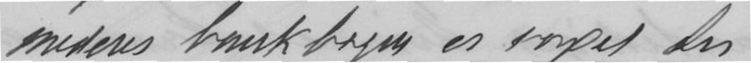medens bankbogen er vogset til
